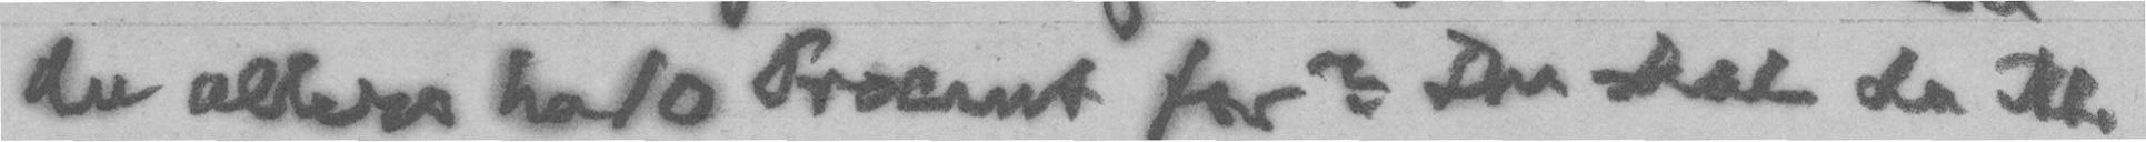du ellers ha 10 Procent for? Du skal da ikke
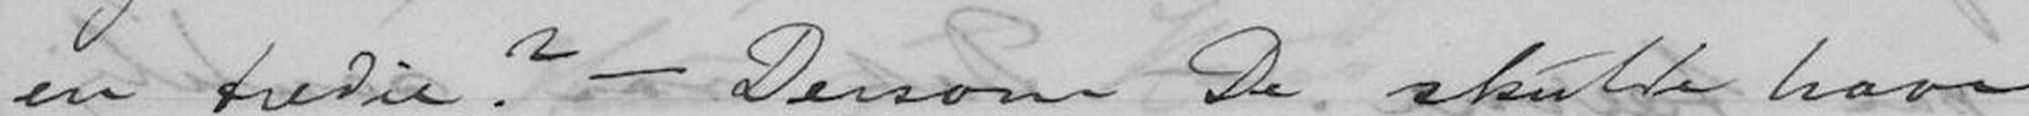en tredie? - Dersom De skulde have
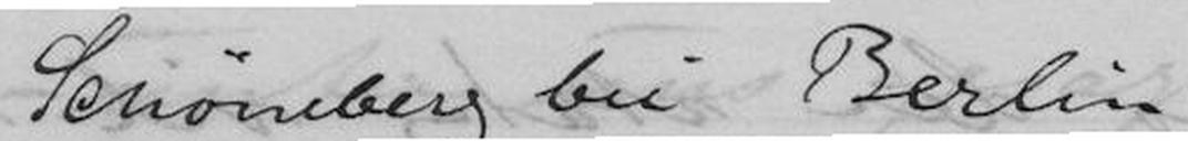Schöneberg bei Berlin
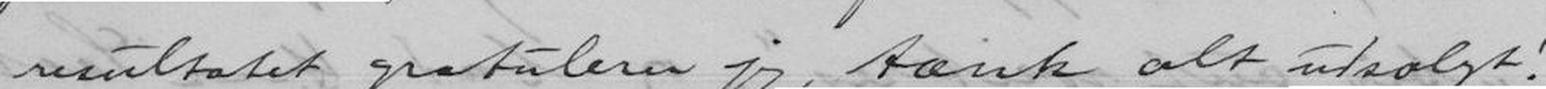resultatet gratulerer jeg, tænk alt udsolgt!
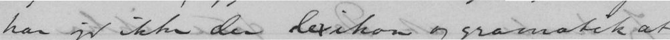har jo ikke den Lexikon og gramatik at
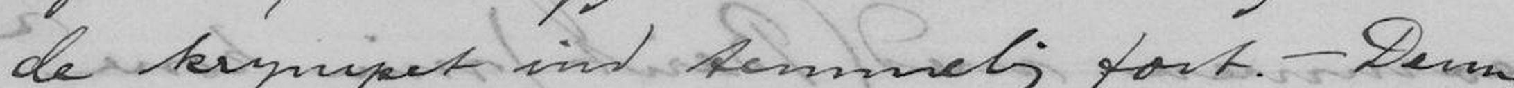de krympet ind temmelig fort. - Denne
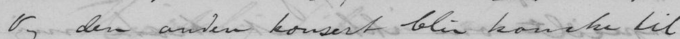Og den anden konsert blir kanske til
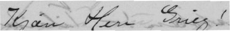Kjære Herr Grieg!
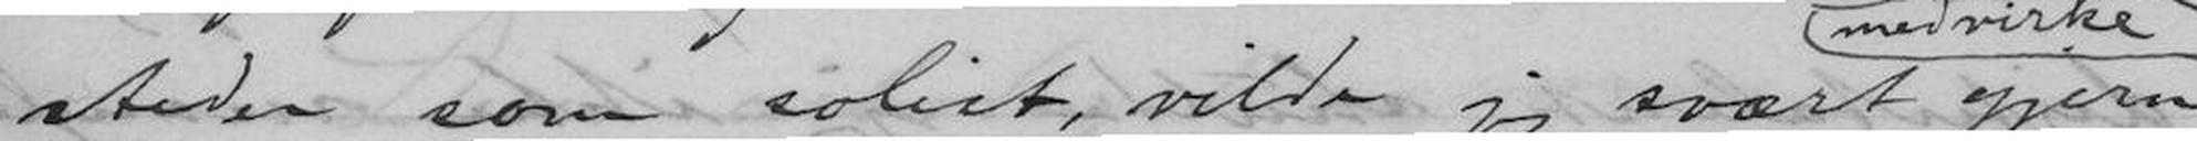steder som solist, vilde jeg svært gjerne
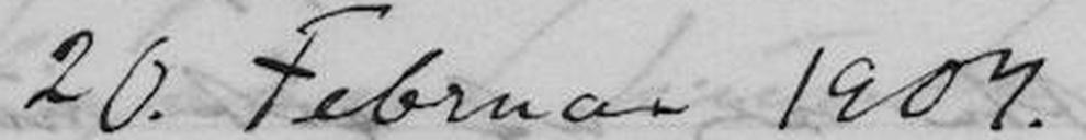20. Februar 1907
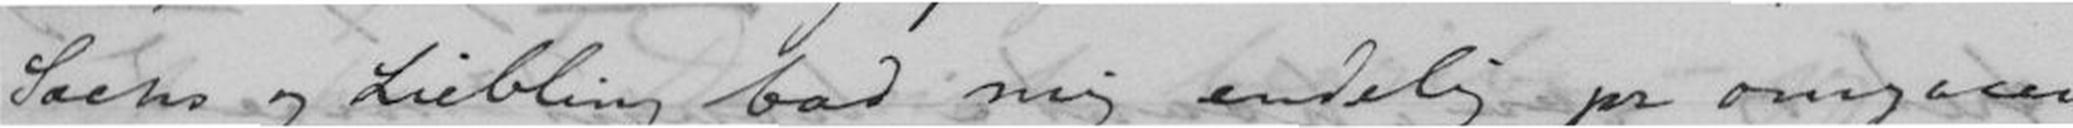Sachs og Liebling bad mig endelig pr. omgaaende
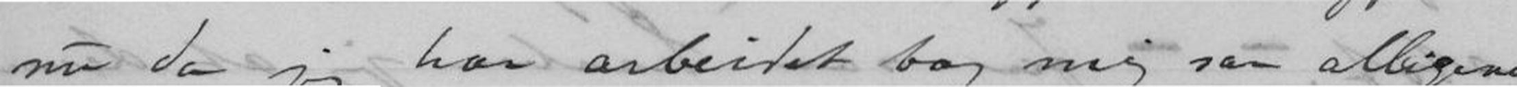nu da jeg har arbeidet bag mig saa alligevel.
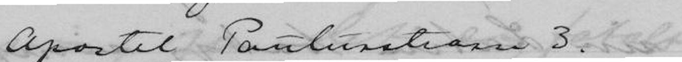Apostel Paulusstrasse 3
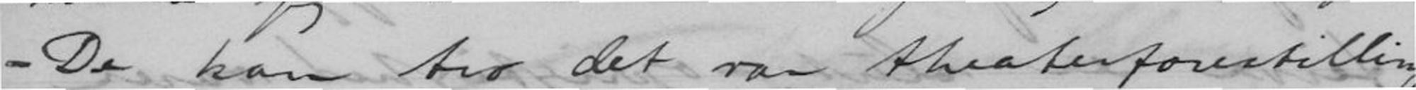- De kan tro det var theaterforestilling
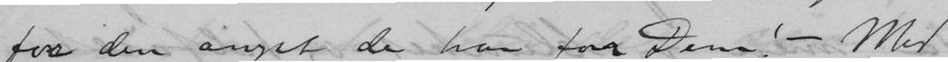for den angst de har for Dem! - Med
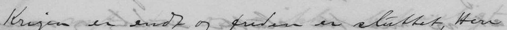Krigen er endt og freden er sluttet, Herr
